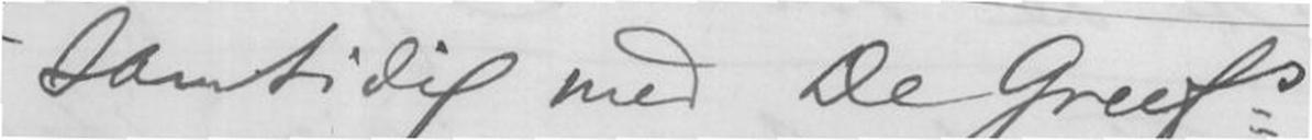x samtidig med De Greefs
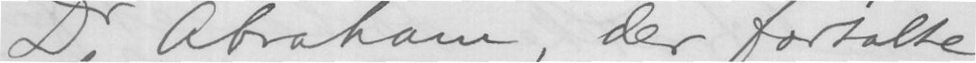Dr Abraham, der fortalte
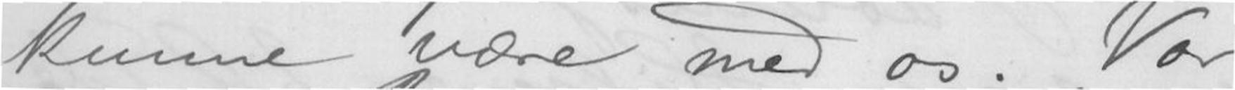kunne være med os. Vor
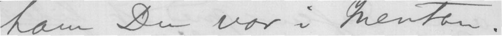ham Du var i Nevton.
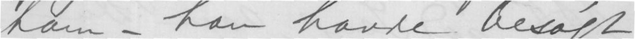ham - han havde besøgt
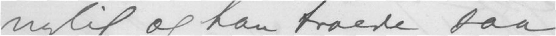nylig og han troede saa
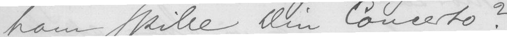ham spille Din Concerto?
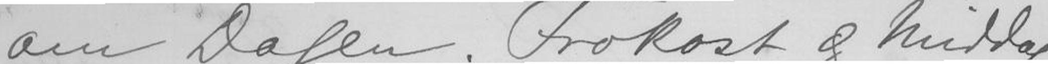om Dagen. Frokost og Middag
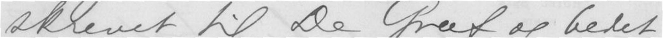skrevet til De Greef og bedet
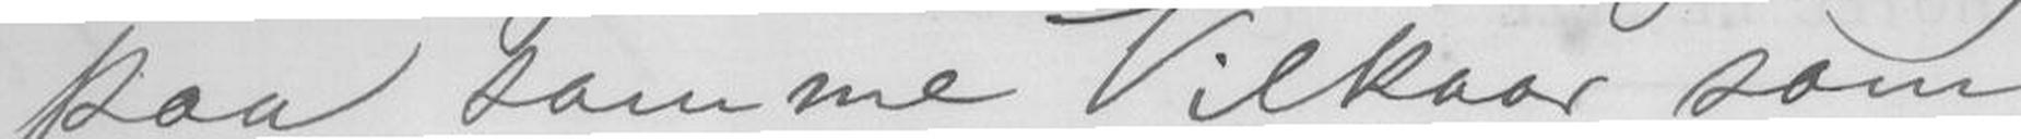paa samme Vilkaar som
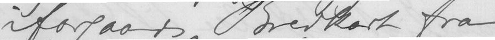iforgaars Brevkort fra
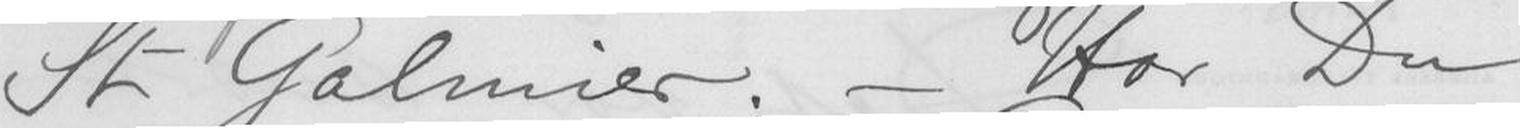St Galmier. - Har Du
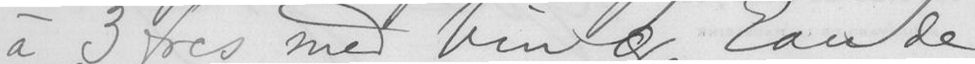à 3 frcs med Vin og Eau de
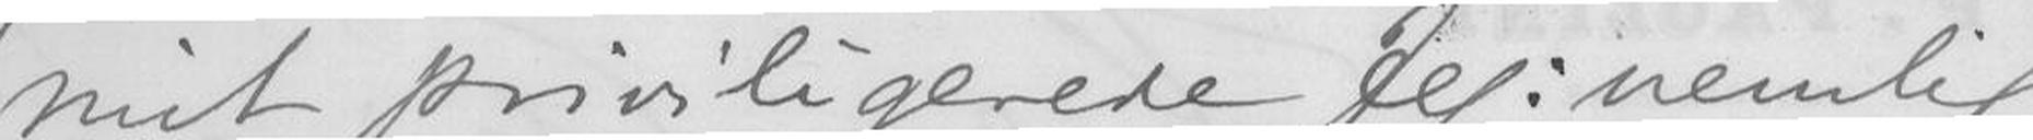mit priviligerede Jeg: nemlig
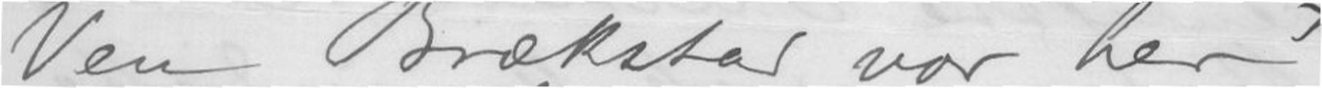Ven Brækstad var herx
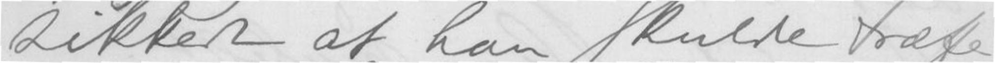sikker at han skulde træffe
